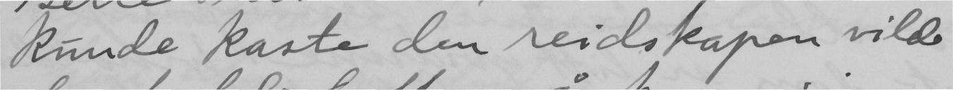kunde kaste den reidskapen vilde
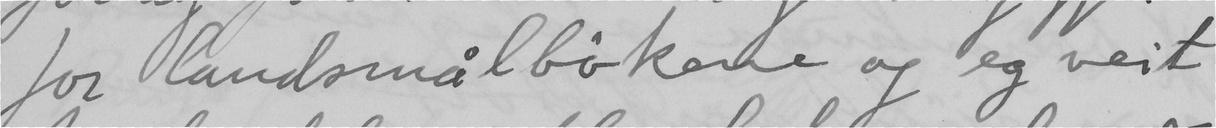for landsmålbökene og eg veit
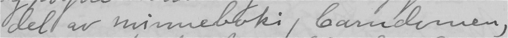del av minneboki, barndomen,
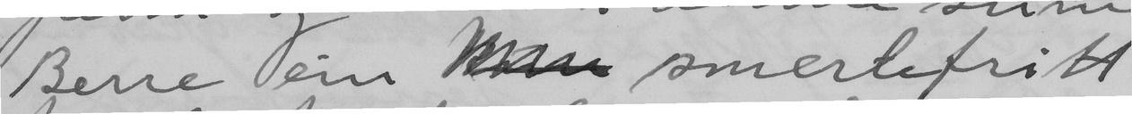Berre ein  smertefritt
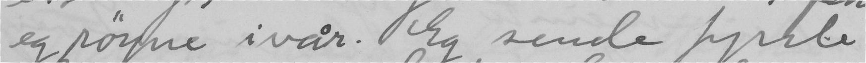eg röyne i vår. Eg sende fyrste
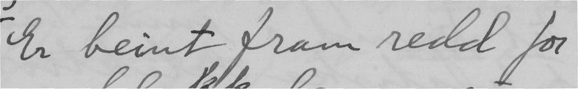Er beint fram redd for
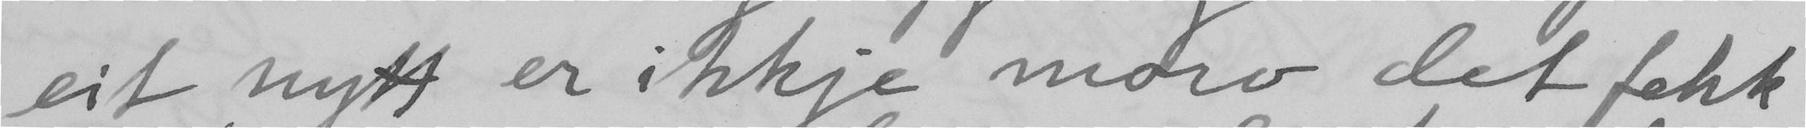eit nytt er ikkje moro det fekk
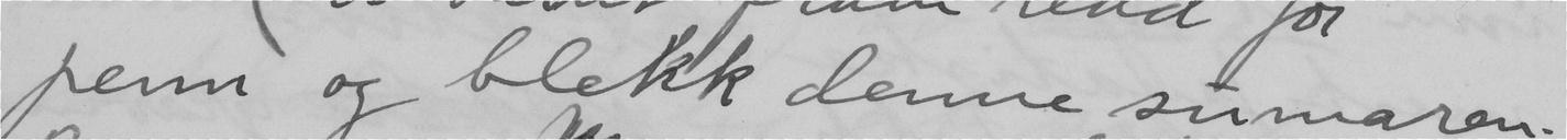penn og blekk denne sumaren.
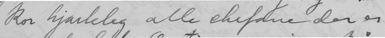kor hjarteleg alle chefene der er
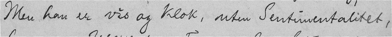Men han er vis og klok, uten Sentimentalitet,
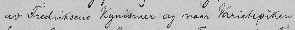av Fredriksens Kynismer og naar Varietepiken
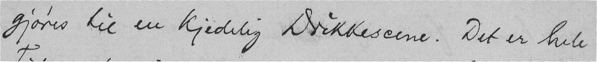gjøres til en kjedelig Drikkescene. Det er hele
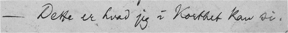- Dette er hvad jeg i Korthet kan si.
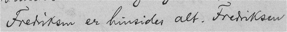Fredriksen er hinsides alt. Fredriksen
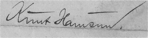Knut Hamsun.
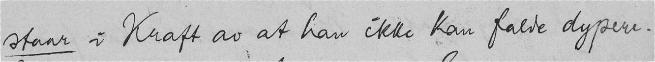staar i Kraft av at han ikke kan falde dypere.
